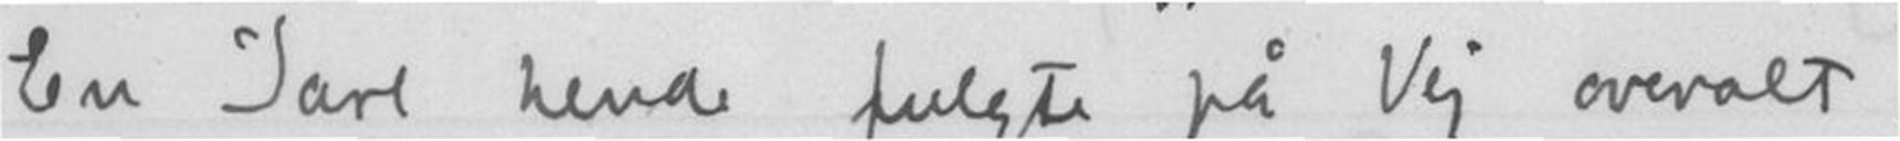En Jarl hende fulgte på Vej overalt,
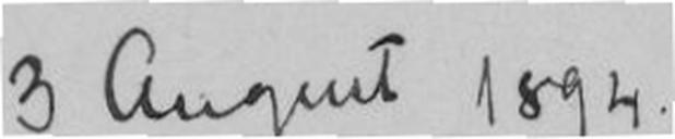3 August 1894.
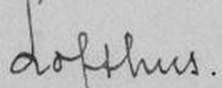Lofthus.
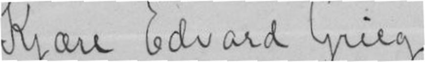Kjære Edvard Grieg.
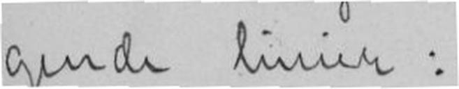gende linier:
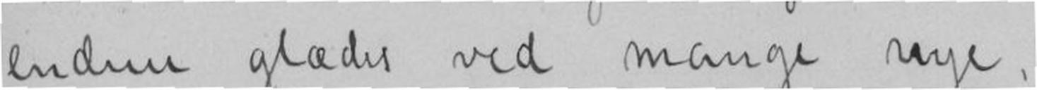endnu glædes ved mange nye,
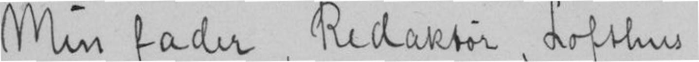Min fader, Redaktør, Lofthus
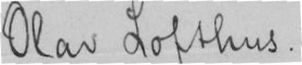Olav Lofthus.
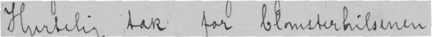Hjertelig tak for blomsterhilsenen
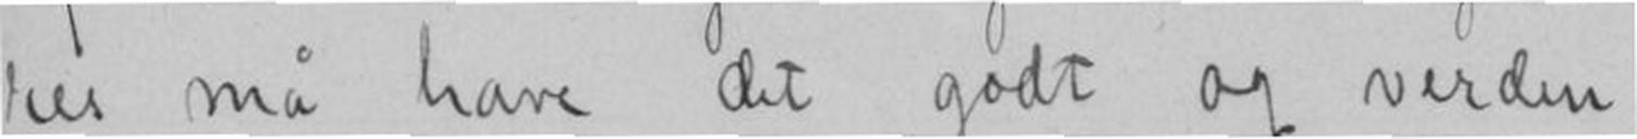res må have det godt og verden
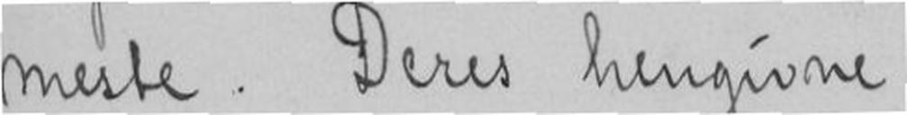meste. Deres hengivne
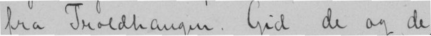fra Troldhaugen. Gid de og de
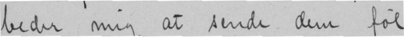beder mig at sende dem følx
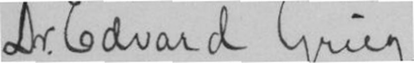Dr. Edvard Grieg !
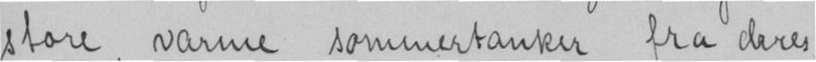store varme sommertanker fra deres
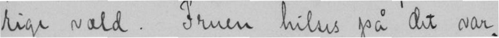rige væld. Fruen hilses på det var-
